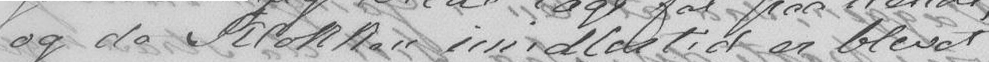og da Klokken imidlertid er blevet
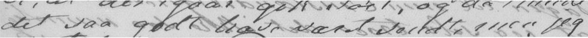det saa godt have været sendt, men jeg
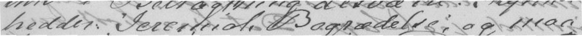hedder Jeremich Bogrædelse, og maa
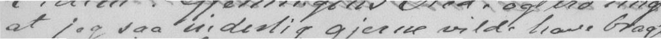at jeg saa inderlig gjerne vilde have bragt
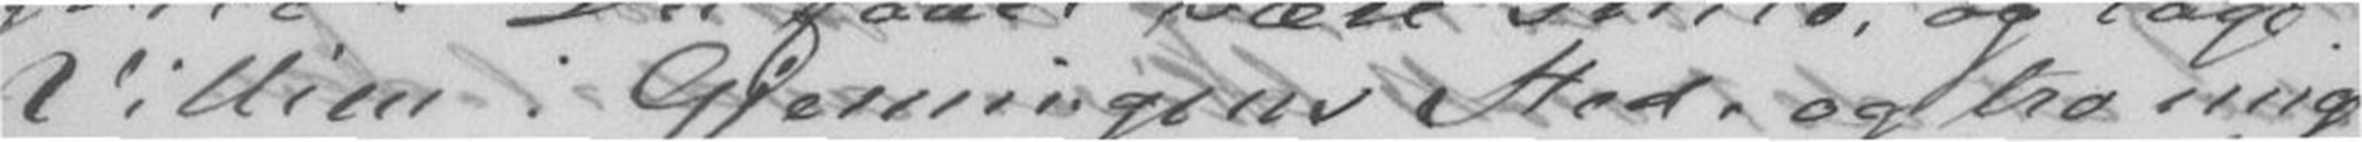Villien. Gjerningens Sted og tro mig
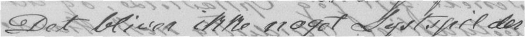Det bliver ikke noget Lystspil der
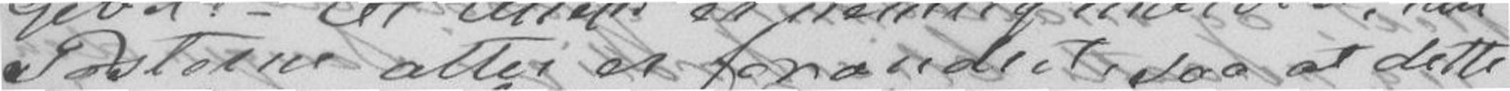Posterne atter er forandret, saa at dette
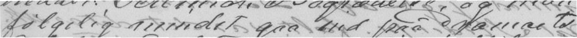følgelig mindst gaa ind paa Dramaets
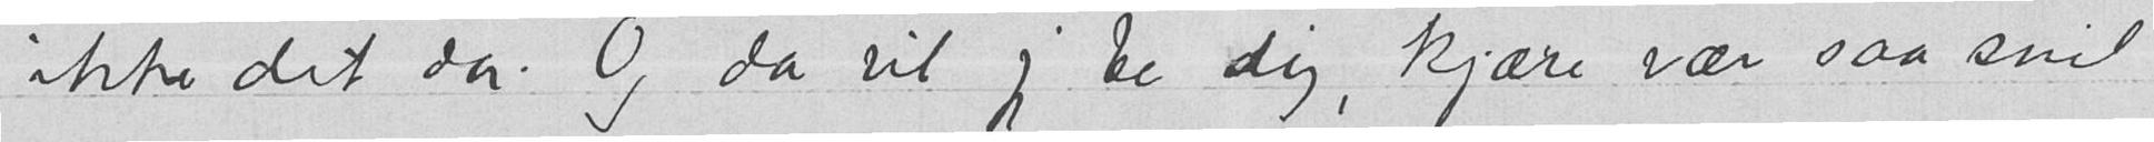ikke det da. Og da vil jeg be dig, kjære vær saa snil
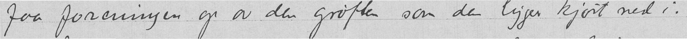faa foreningen op av den grøften som den ligger kjørt ned i.
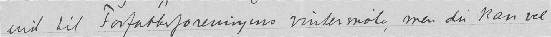ind til Forfatterforeningens vintermøte, men du kan vel
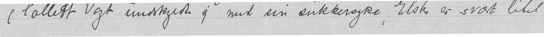(Collett Vogt undskyldte sig med sin sukkersyke, Elster er svært litet
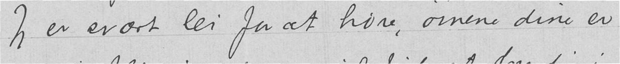Jeg er svært lei for at høre, øinene dine er
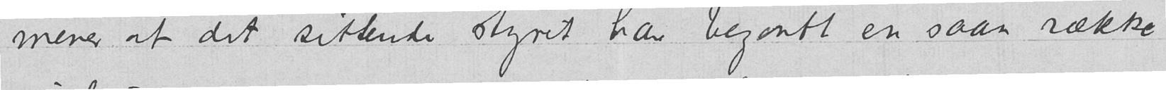mener at det sittende styret har begaatt en saan række
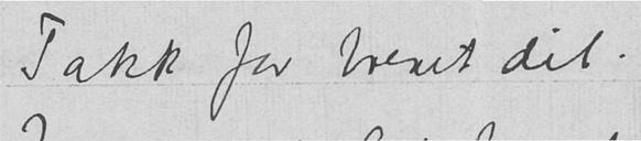Takk for brevet dit.
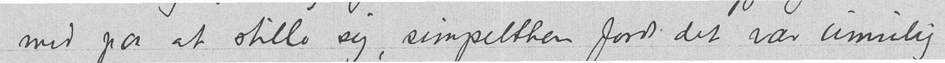med paa at stille sig, simpelthen fordi det var umulig
